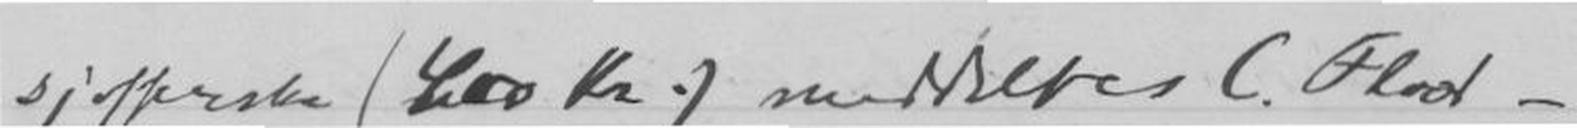sjofferske (400 Kr.) meddel.es C. Flod -
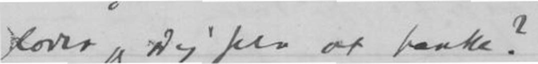lader jeg Dig selv at tænke?
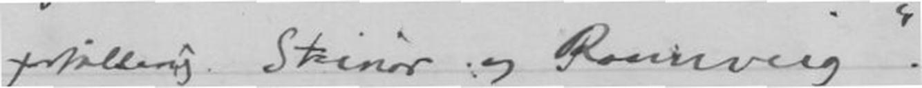fortælling "Steiner og Rannveig".
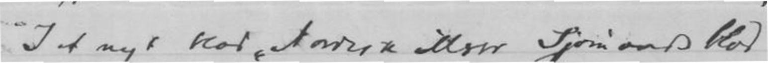I et nyt blad "Nordisk M... Sjømandsblad"
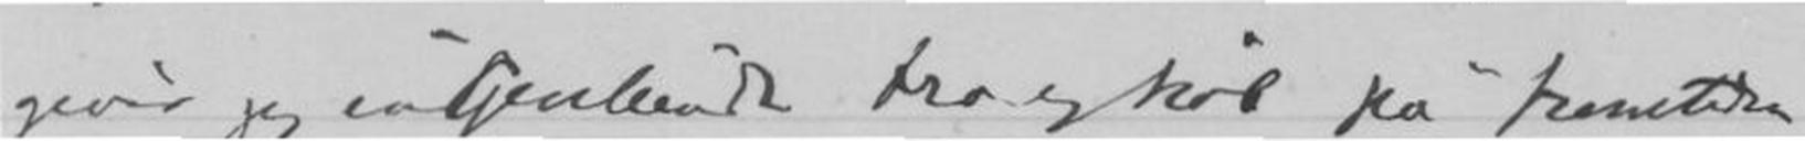giver jeg indtjenlunde tro og håb på fremtiden
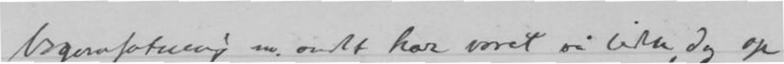gernsætning en andet har været så liten, dog op
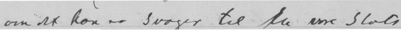om det han er svoger til En vore Stats
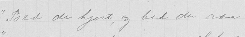"Bed du hjort, og bed du raa
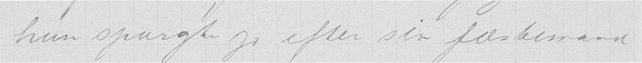hun spurgte jo efter sin fæstemand
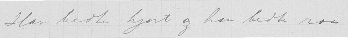Han bedte hjort og han bedte raa
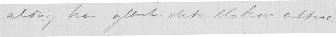aldrig han glemte den elskovs attraa.
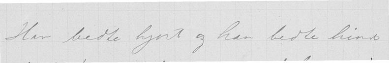Han bedte hjort og han bedte hind
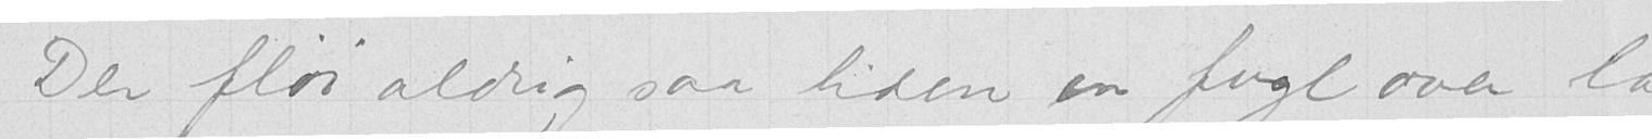Der fløi aldrig saa liden en fugl over land
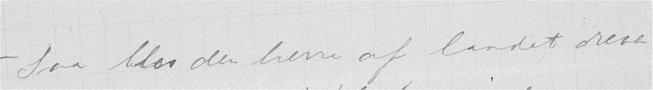— Saa blev den herre af landet dreven
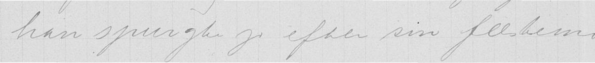han spurgte jo efter sin fæstemø.
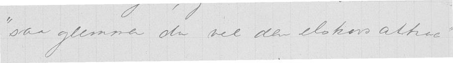"saa glemmer du vel den elskovs attraa"
